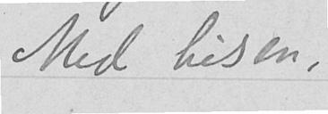Med hilsen,
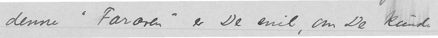denne "Furoren" er De snil, om De kunde
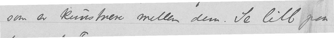som er kunstnere mellem dem. Se litt paa
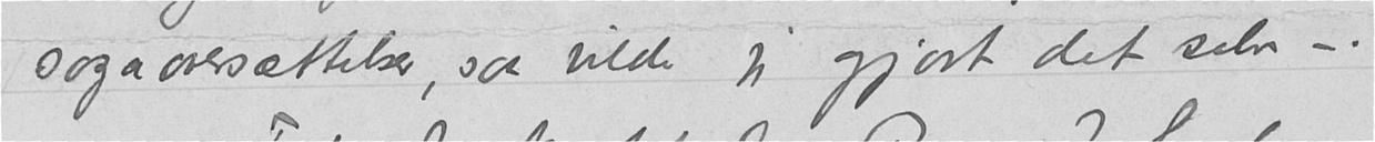sagaoversættelser, saa vilde jeg gjort det selv –.
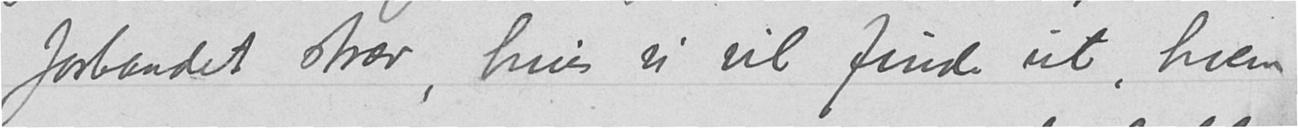forbandet stræv, hvis vi vil finde ut, hvem
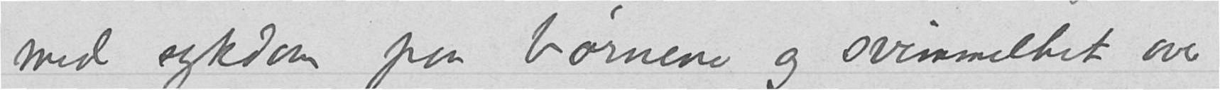med sykdom paa børnene og svimmelhet over
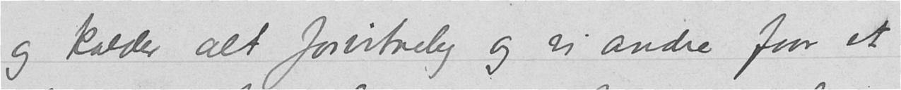og kalder alt forvitnelig og vi andre faar et
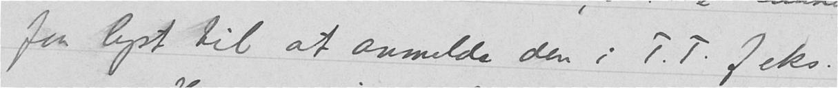faa lyst til at anmelde den i T.T. f eks.
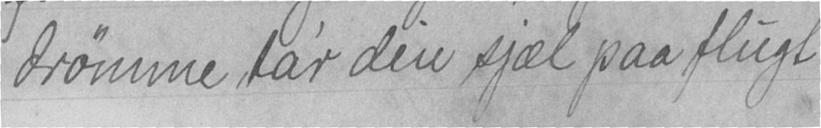drømme tar den sjæl paa flugt
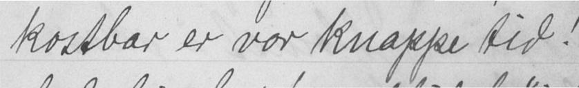kostbar er vor knappe tid!
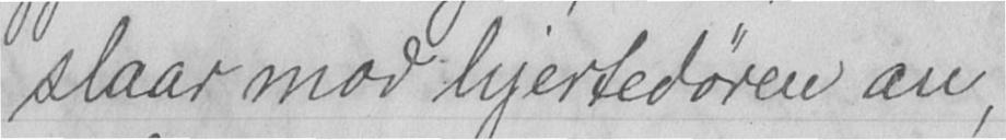slaar mod hjertedøren an,
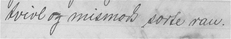tvivl og mismods sorte ran.
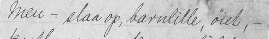Men - slaa op, barnlille, øiet, -
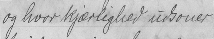og hvor kjærlighed udsoner
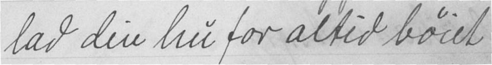lad din hu for altid bøiet
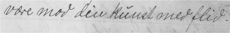være mod din kunst med flid.
